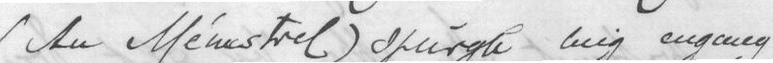(Tu spurgte mig engang,
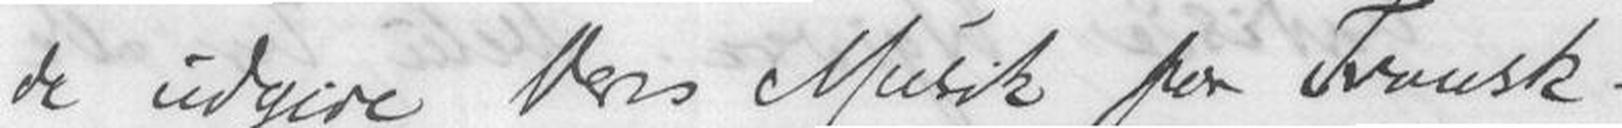de udgive Deres Musik for Fransk.
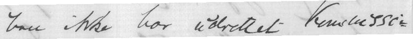han ikke har udrettet Konsklussi-
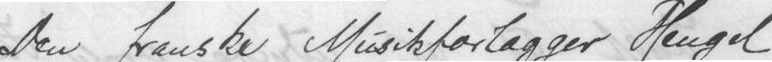Den franske Musikforlægger Heugel
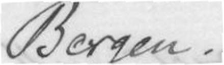Bergen
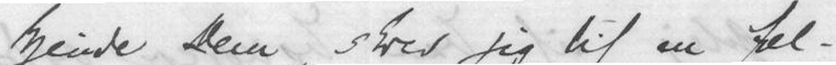kjende Dem, skrev jeg til en fæl-
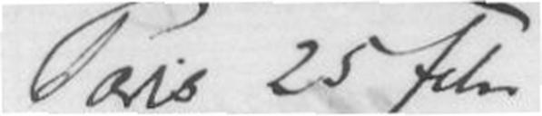Paris 25febr.
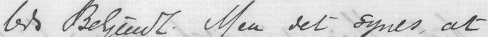les betjendt. Men det synes, at
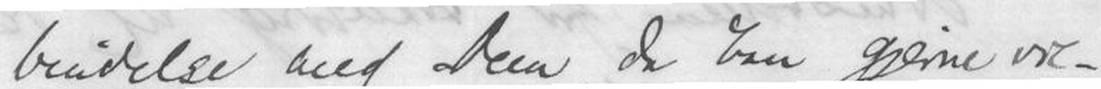bindelse med Dem, da han gjerne vil-
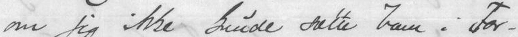om jeg ikke burde sætte ham i For-
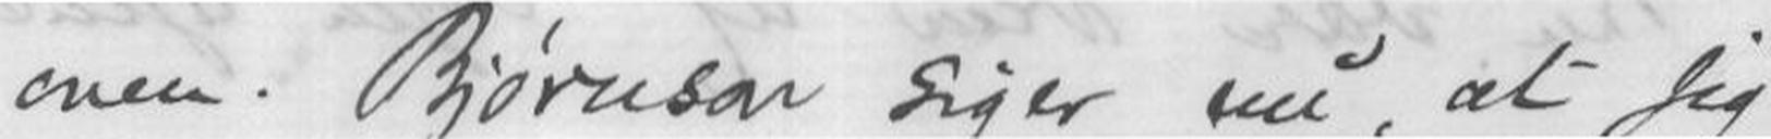onen. Bjørnson siger nu, at jeg
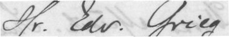Hr. Edv Grieg!
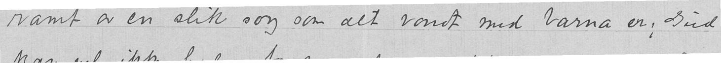ramt av en slik sorg som alt vondt med barna er; Gud
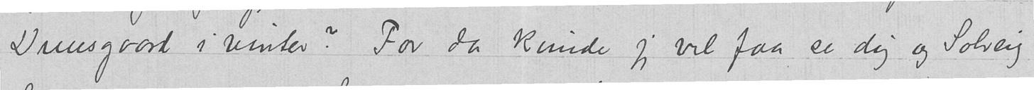Duusgaard i vinter? For da kunde jeg vel faa se dig og Solveig
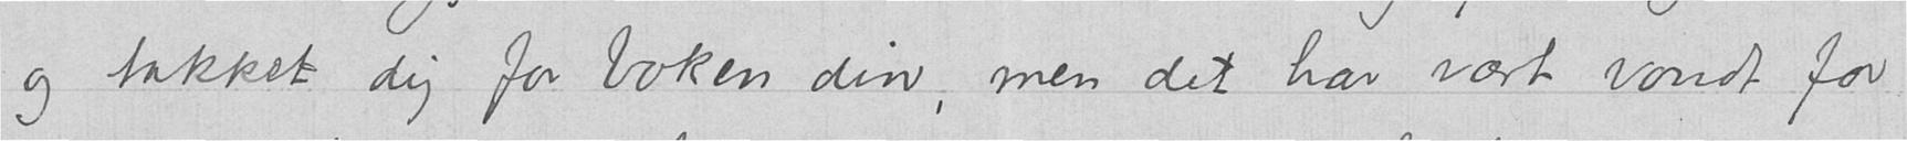og takket dig for boken din, men det har vært vondt for
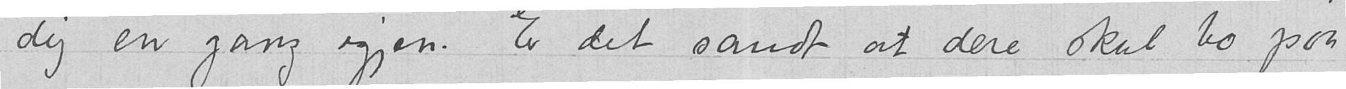dig en gang igjen. Er det sandt at dere skal bo paa
